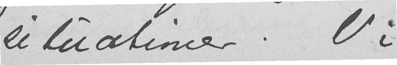situationer. Vi
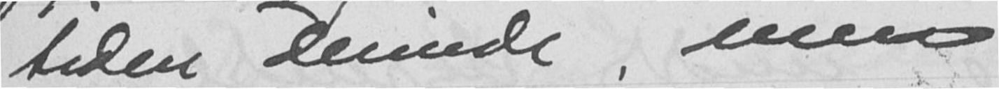tiden derinde, men
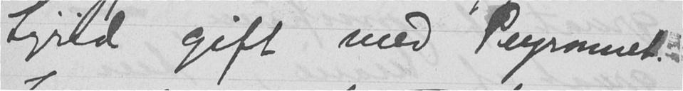Sigrid gift med Peyronnet.
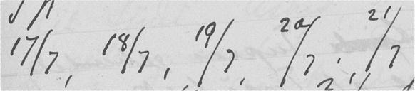17/7, 18/7, 19/7, 20/7, 21/7
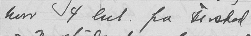hvor 4 lnt. fra Fr.stad
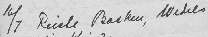16/7 Reiste Basken, Wedels
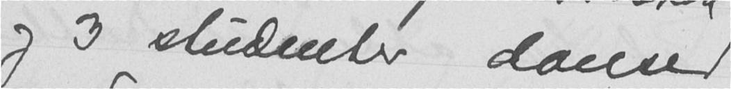og 3 studenter dansed
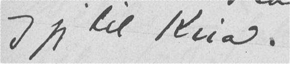og jeg til Kria.
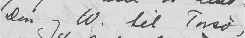Don og W. til Torsø,
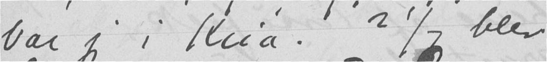var jeg i Kria. 21/7 blev
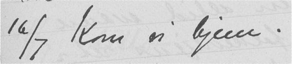16/7 Kom vi hjem.
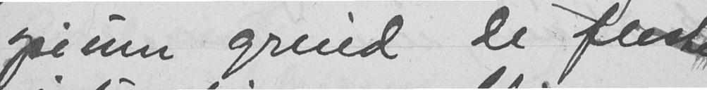opium greied de fleste
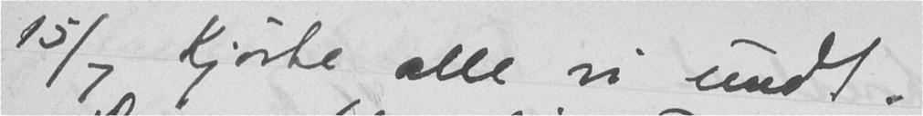15/3 Kjørte alle vi rundt.
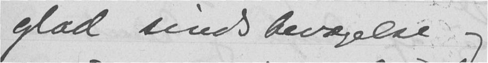glad sindsbevægelse og
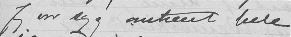Jeg var syg omtrent hele
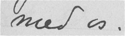med os.
